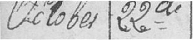October 22de
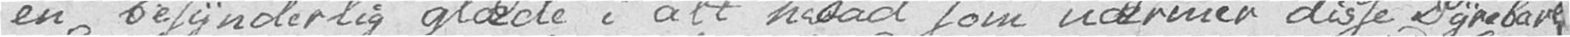en besynderlig glæde i alt hvad som nærmer disse Dyrebare
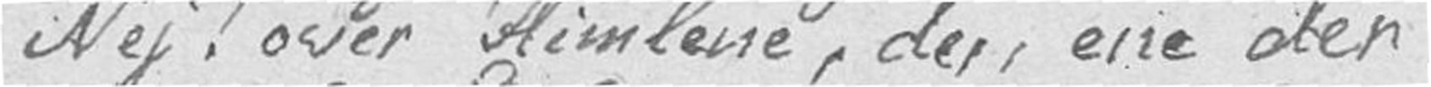Nej! over Himlene, der, ene der
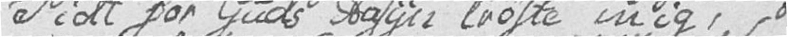Tidt for Guds Aasyn trøste mig,
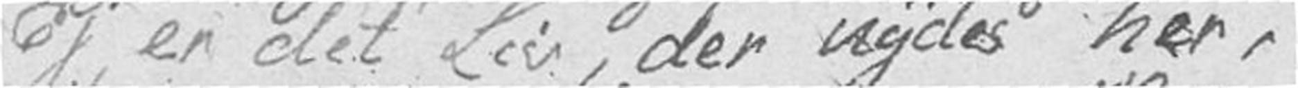Ej er det Liv, der nydes her,
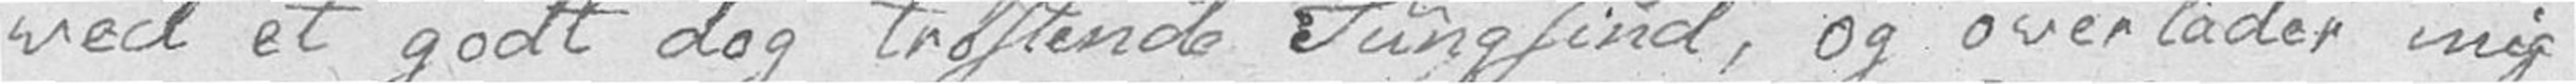ved et godt dog trøstende Tungsind, og overlader mig
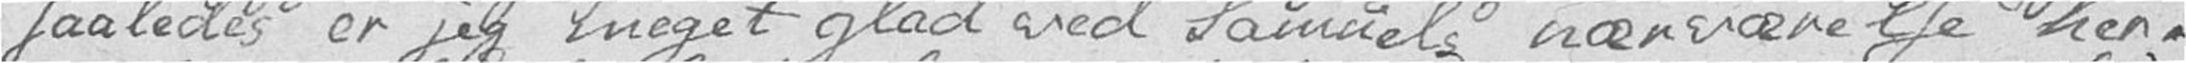saaledes er jeg meget glad ved Samuels nærværelse her.
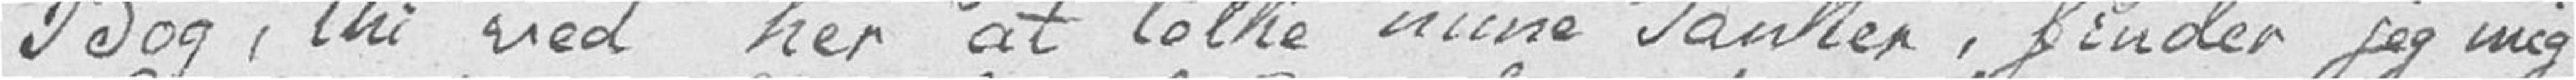Bog, thi ved her at tolke minne Tanker, finder jeg mig
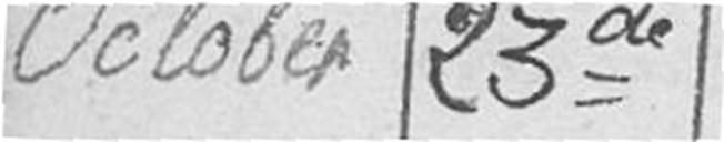October 23de
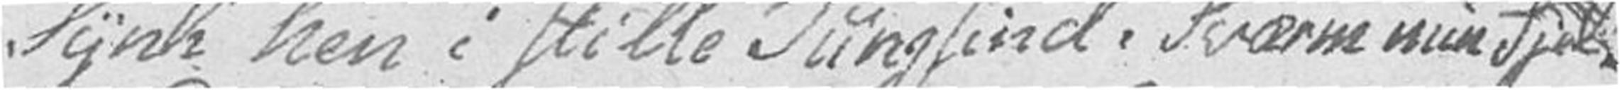Synk hen i stille Tungsind! Sværm min Sjel.
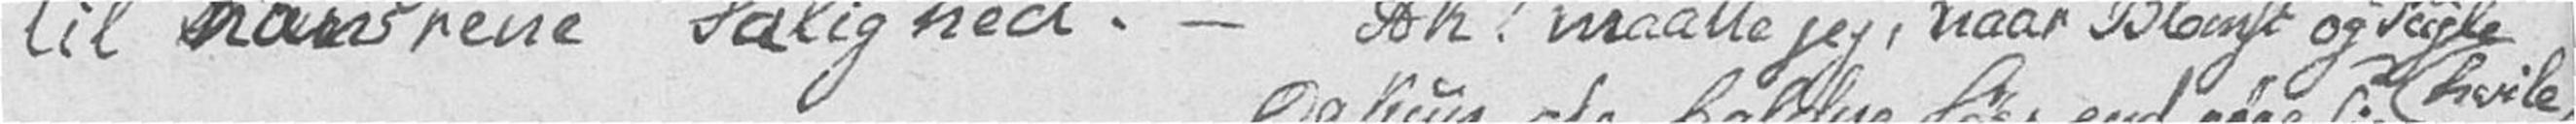til hans rene Salighed. – Ak! maatte jeg, naar Blomst og Fugle hvile
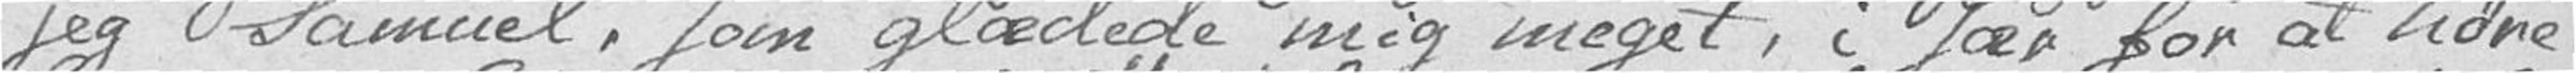jeg Samuel, som glædede mig meget, i sær for at høre
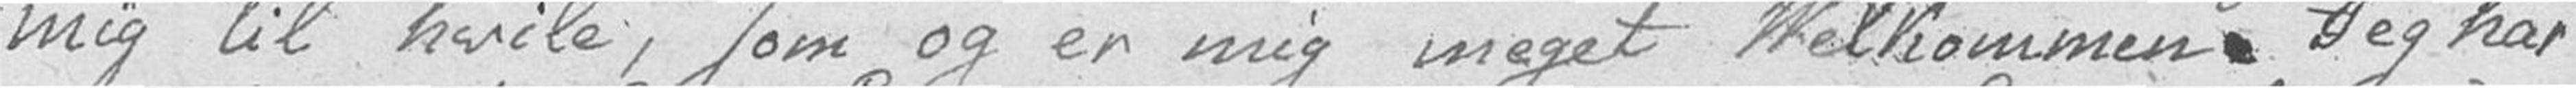mig til hvile, som og er mig meget Velkommen. Jeg har
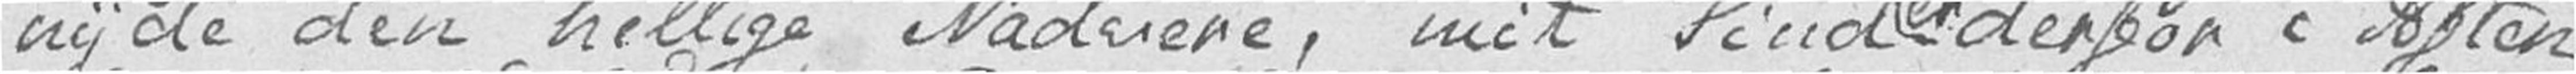nyde den hellige Nadvere, mit Sind er derfor i Aften
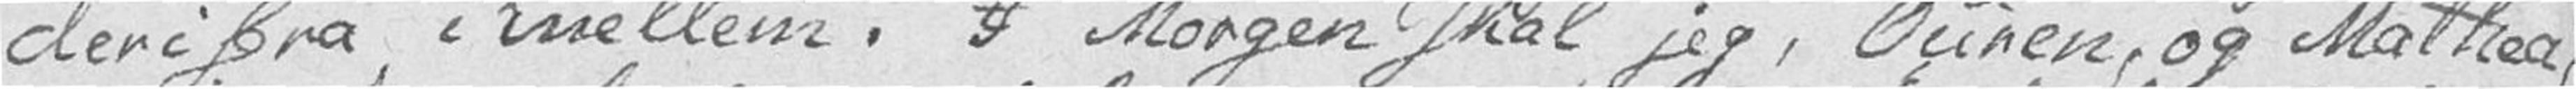derifra imellem. I Morgen skal jeg, Ouren, og Mathea,
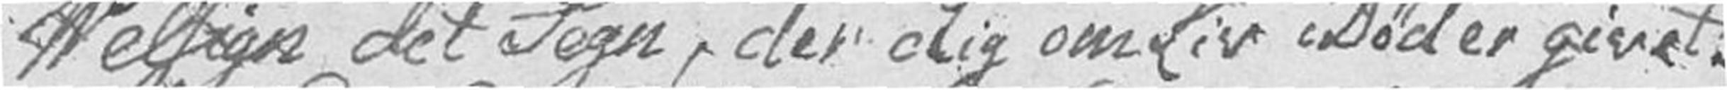Velsign det Tegn, der dig om Liv i Død er givet.
